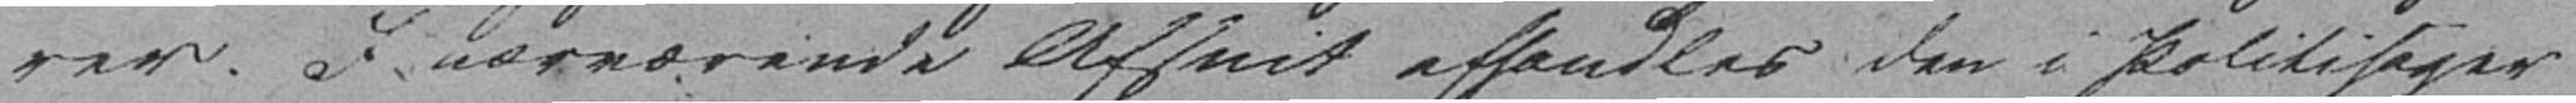rer. I nærværende Afsnit afhandles den i Politisager
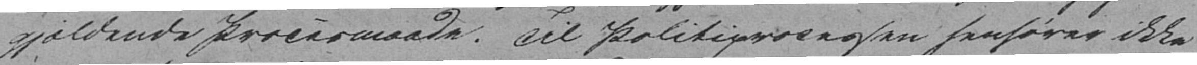gjældende Procesmaade. Til Politiprocessen henhører ikke
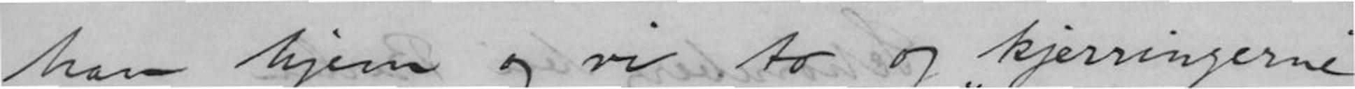han hjem og vi to og "kjerringerne"
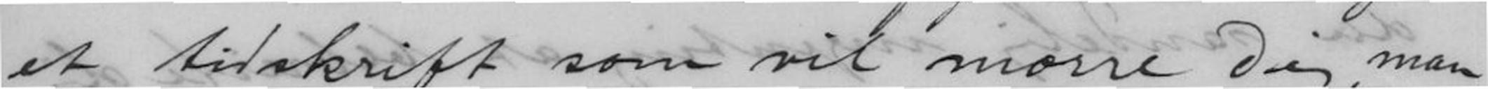et tidskrift som vil morre Dig, man
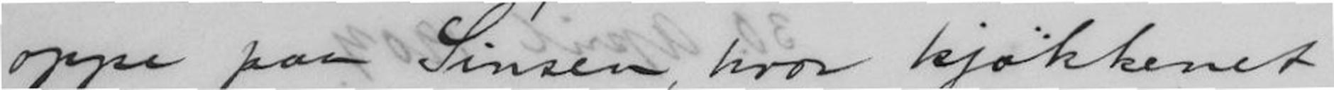oppe paa Sinsen, hvor kjøkkenet
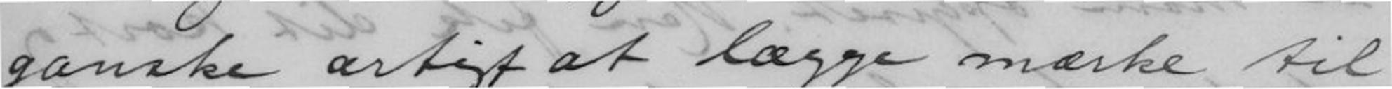ganske artig at lægge mærke til
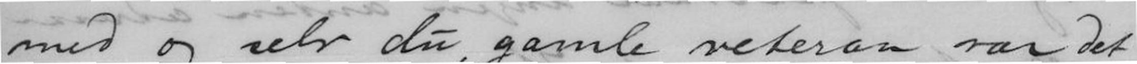med og selv du, gamle veteran var det
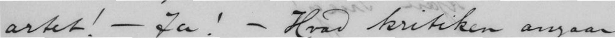artet! - Ja! - Hvad kritiken angaar
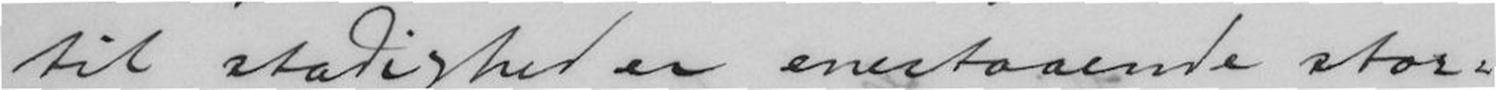til stadighed er enestaaende stor-
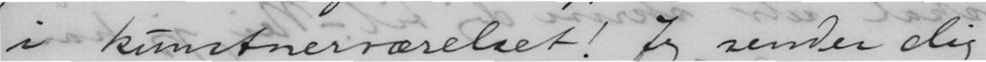i kunstnerværelset! Jeg sender dig
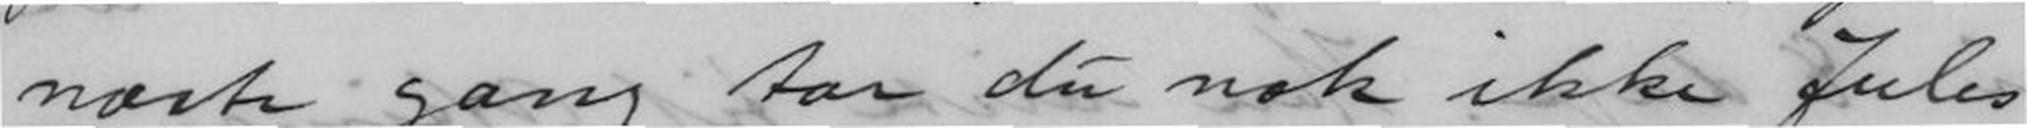næste gang tar du nok ikke Jules
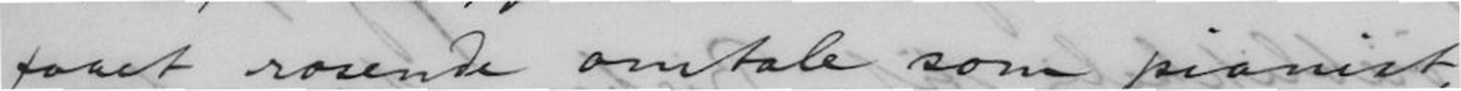faaet rosende omtale som pianist,
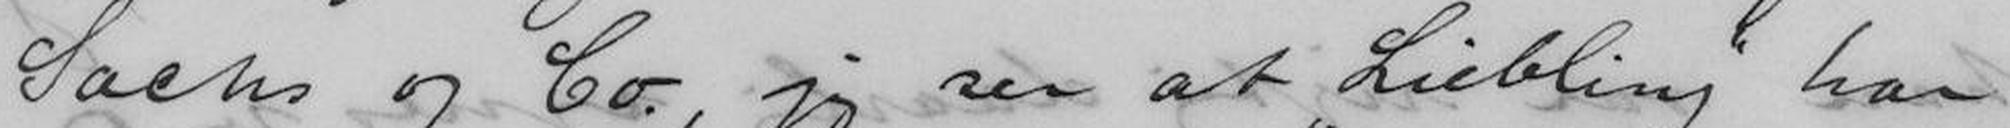Sachs og Co, jeg ser at "Liebling" har
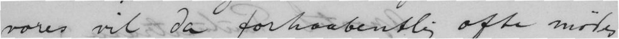vores vil da forhaabentlig ofte mødes
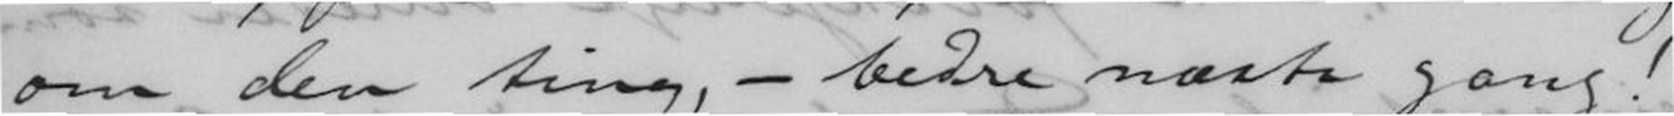om den ting, - bedre næste gang!
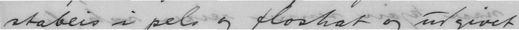stabeis i pels og floshat og udgivet
